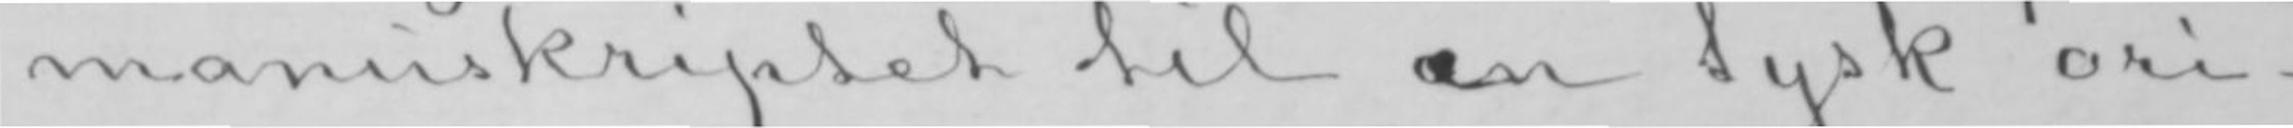manuskriptet til en tysk ori-
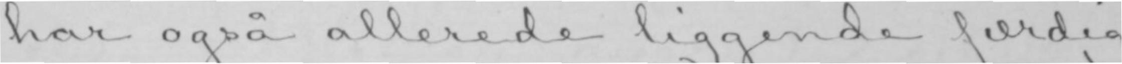har også allerede liggende færdig
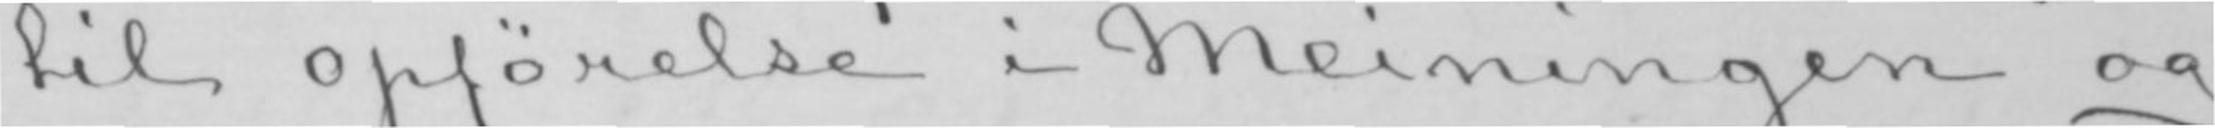til opførelse i Meiningen og
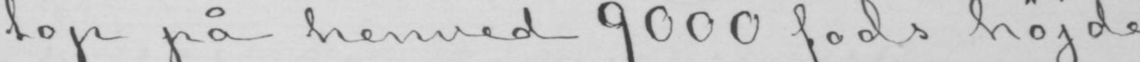top på henved 9000 fods højde,
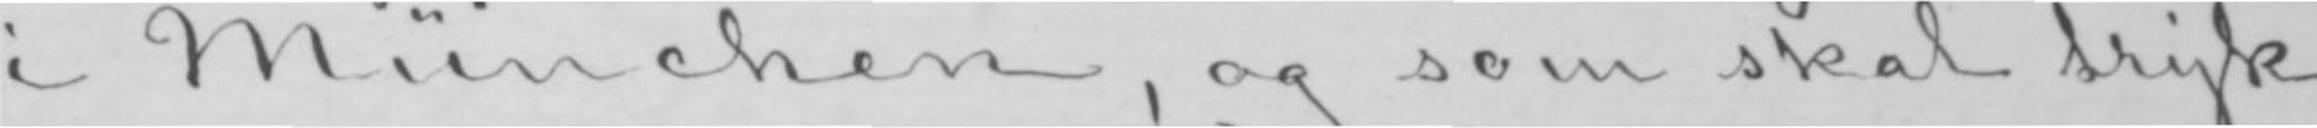i München, og som skal tryk-
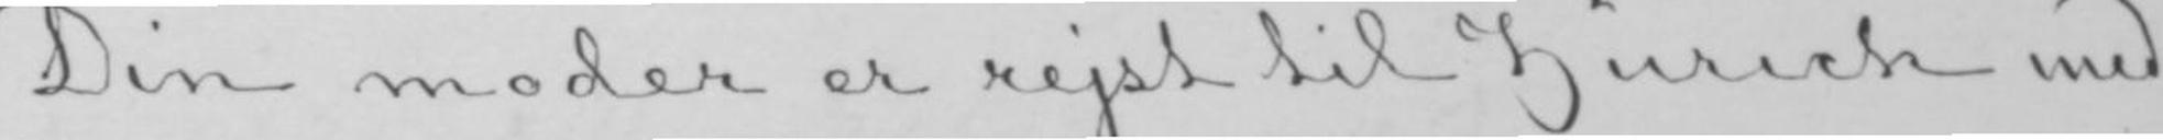Din moder er rejst til Zürich med
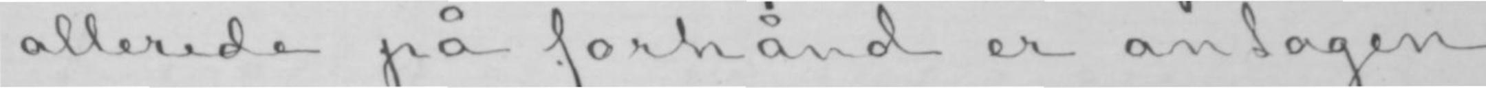allerede på forhånd er antagen
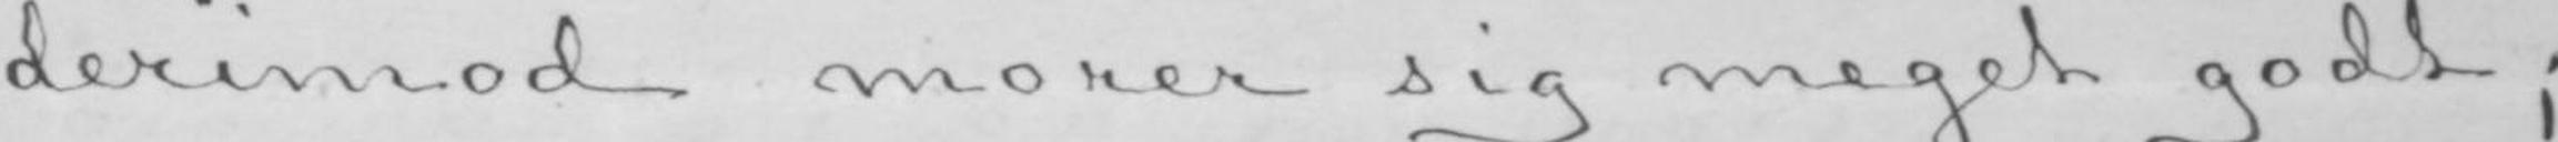derimod morer sig meget godt;
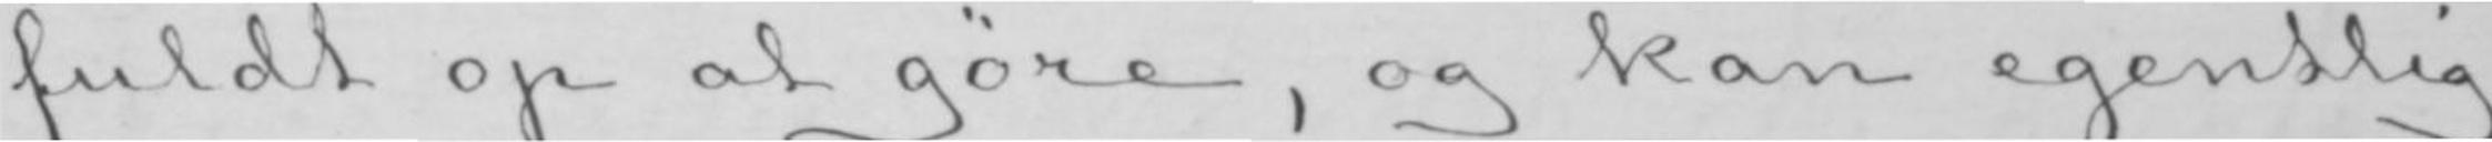fuldt op at gøre, og kan egentlig
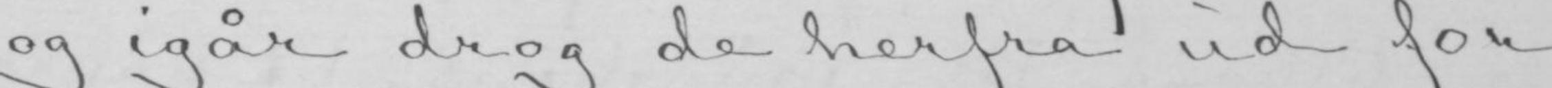og igår drog de herfra ud for
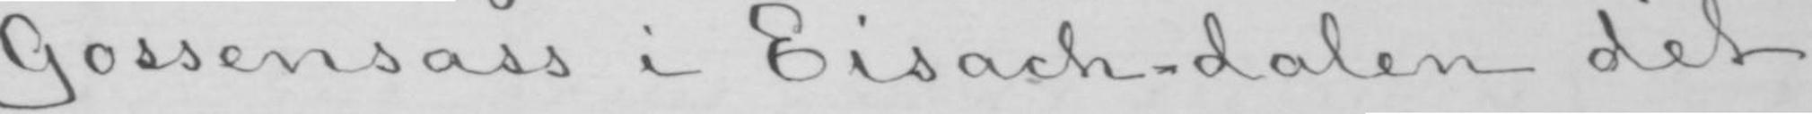Gossensass i Eisach-dalen det
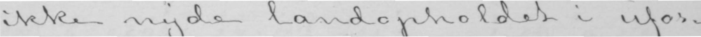ikke nyde landopholdet i ufor-
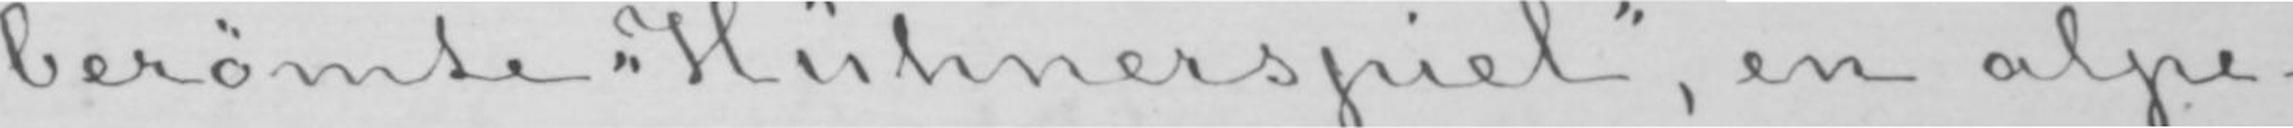berømte «Hühnerspiel», en alpe-
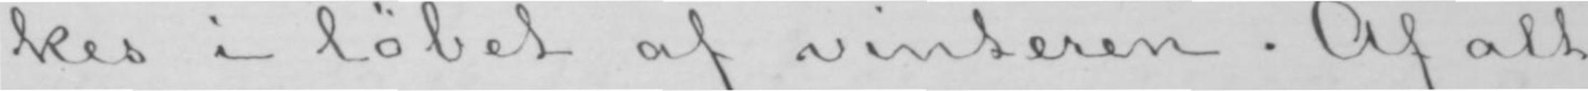kes i løbet af vinteren. Af alt
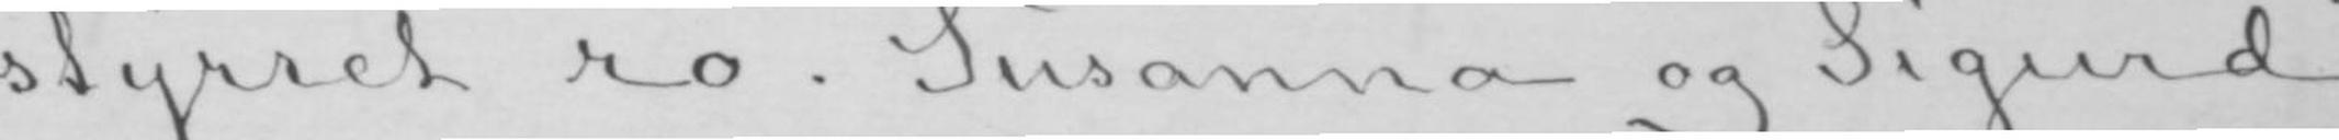styrret ro. Susanna og Sigurd
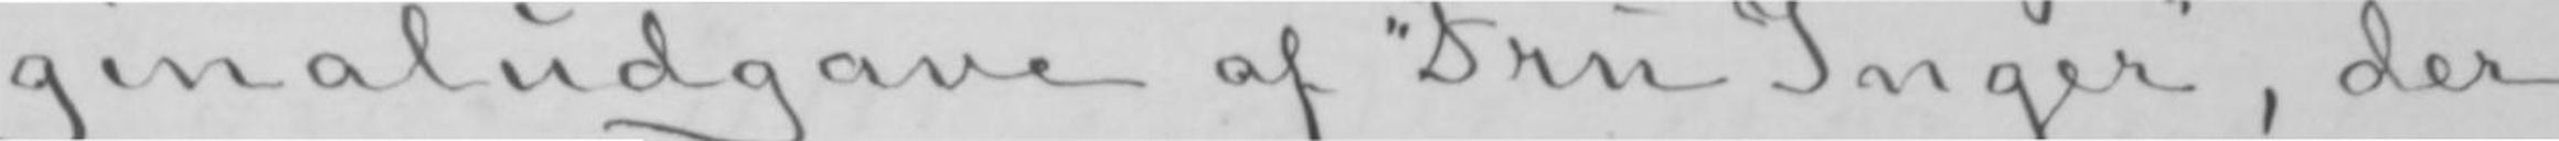ginaludgave af «Fru Inger», der
